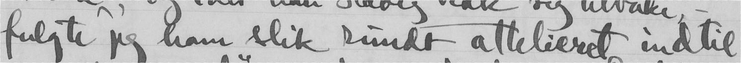fulgte jeg ham slik rundt attelieret indtil
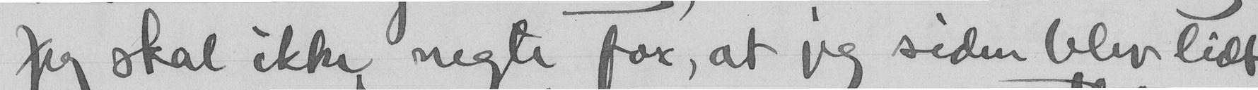Jeg skal ikke negte for, at jeg siden blev lidt
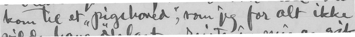kom til et "pigehoved", som jeg for alt ikke
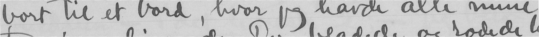bort til et bord hvor jeg havde alle mine
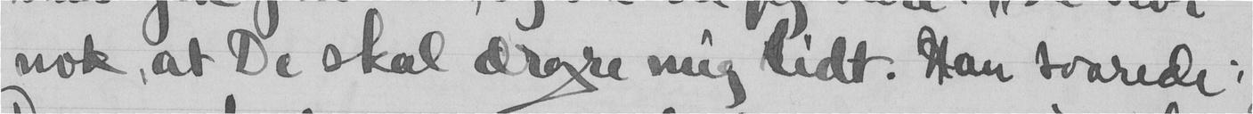nok, at De skal ærgre mig lidt. Han svarede:
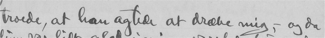troede, at han agtede at dræbe mig,- og da
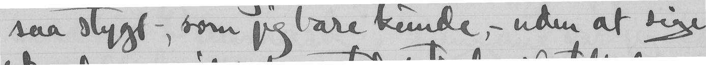saa stygt , som jeg bare kunde, - uden at sige
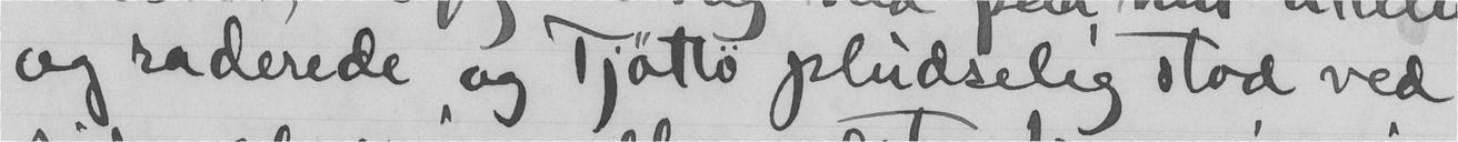og raderede og Tjøttø pludselig stod ved
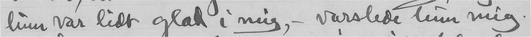hun var lidt glad i mig, - varslede hun mig.
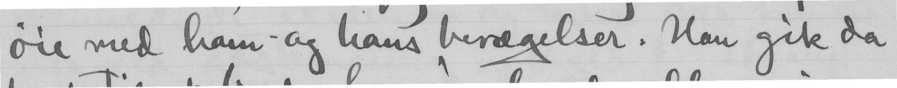øie med ham - og hans bevægelser. Han gik da
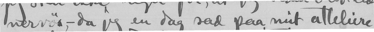nærvøs, - da jeg en dag sad paa mit atteliere
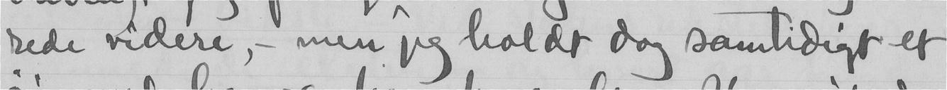rede videre, - men jeg holdt dog samtidigt et
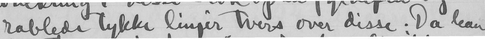rablede tykke linjer tvers over disse. Da han
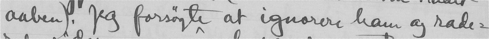aaben). Jeg forsøgte at ignorere ham og rade-
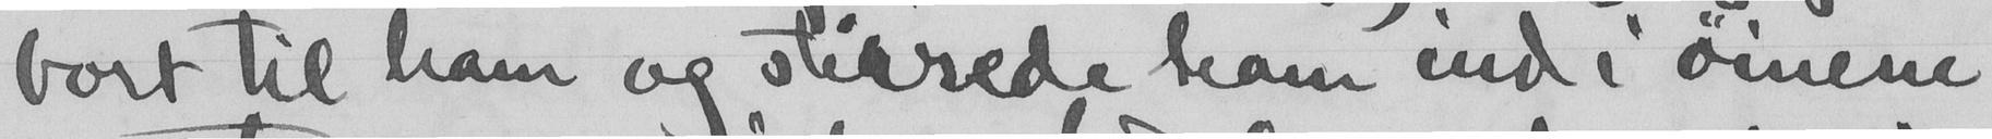bort til ham og stirrede ham ind i øinene
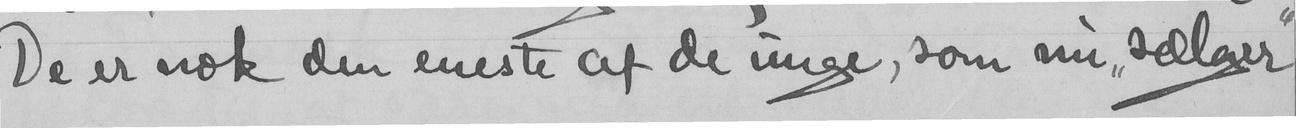De er nok den eneste af de unge, som nu "sælger"
